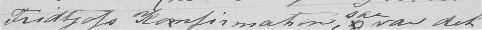Fridtjofs Komfirmation, saa var det
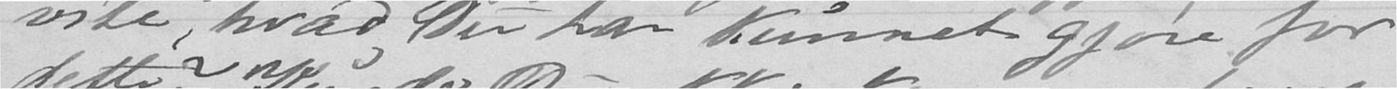vite, hvad Du har kunnet gjøre for
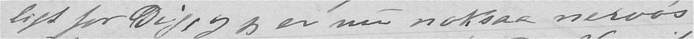ligt for Dig, og jeg er nu noksaa nervøs
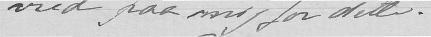vred paa mig for dette.
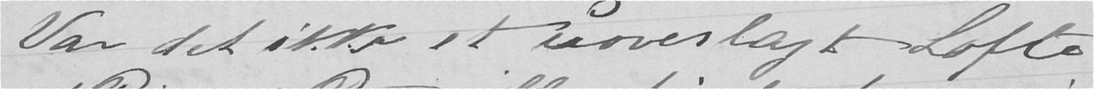Var det ikke et uoverlagt Løfte
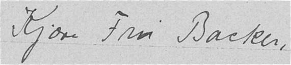Kjære Fru Backer,
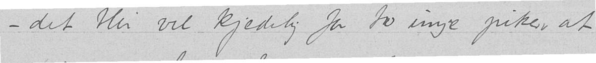– det blir vel kjedelig for to unge piker, at
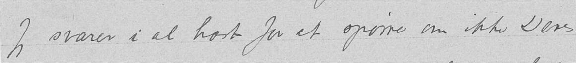Jeg svarer i al hast for at spørre om ikke Deres
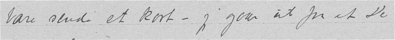bare sende et kort – jeg gaar ut fra at De
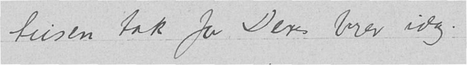tusen tak for Deres brev idag.
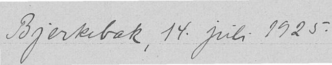Bjerkebæk, 14. juli 1925.
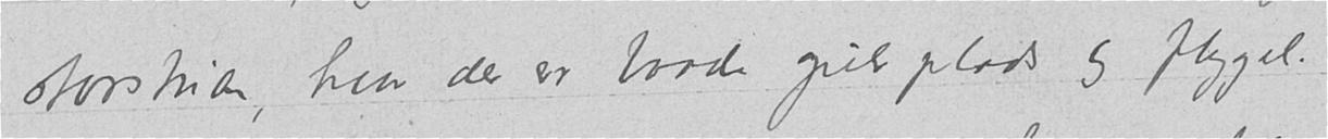storstuen, hvor der er baade gulvplads og flygel.
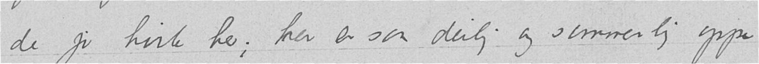de jo hvile her; her er saa deilig og sommerlig oppe
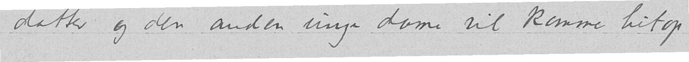datter og den anden unge dame vil komme hitop
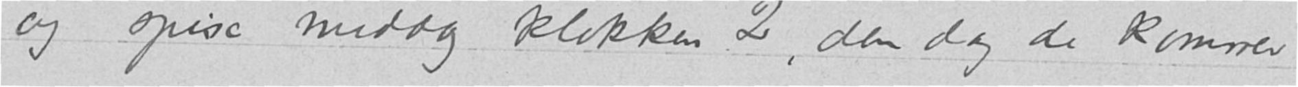og spise middag klokken 2, den dag de kommer
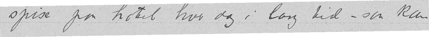spise paa hotel hver dag i lang tid – saa kan
